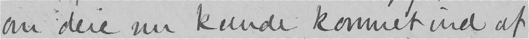om dere nu kunde kommet ind af
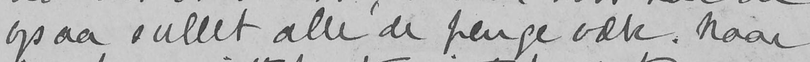ogsaa sullet alle de penge væk. Naar
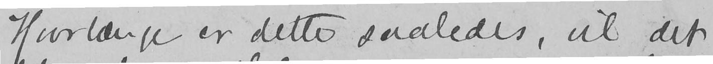Hvorlænge er dette saaledes, vil det
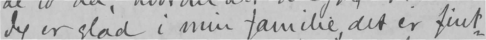Jeg er glad i min familie, det er fint-
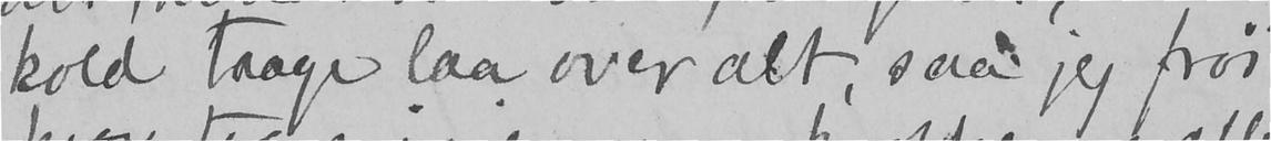kold taage laa over alt, saa jeg frøs
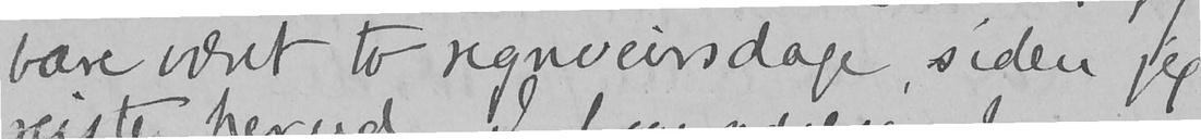bare været to regnveirsdage, siden jeg
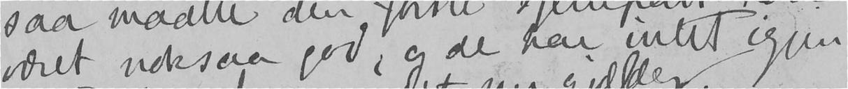været noksaa god, og de har intet igjen
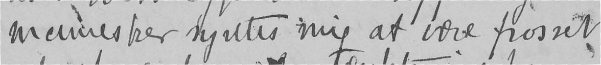mennesker syntes mig at være frosset
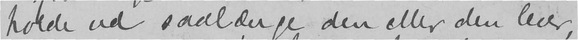holde ud saalænge den eller den lever,
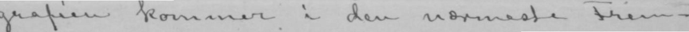grafien kommer i den nærmeste Frem-
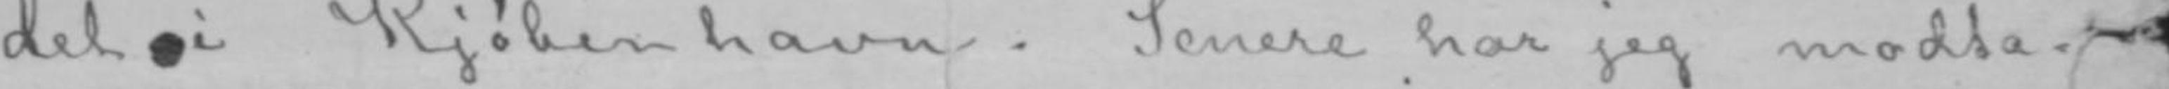det i Kjøbenhavn. Senere har jeg modta-
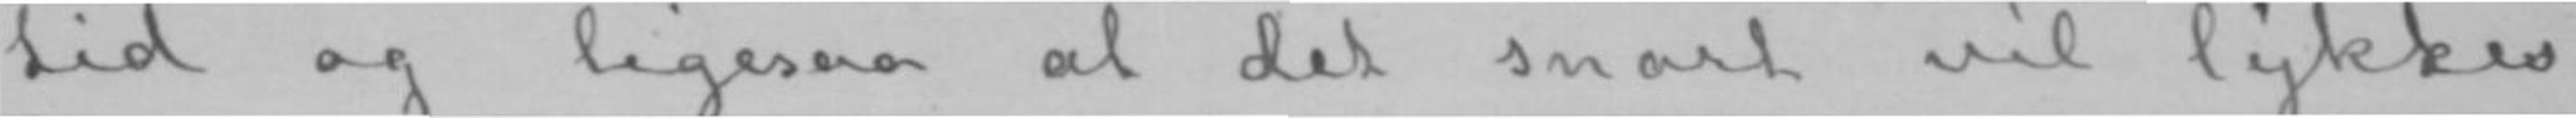tid og ligesaa at det snart vil lykkes
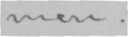men.
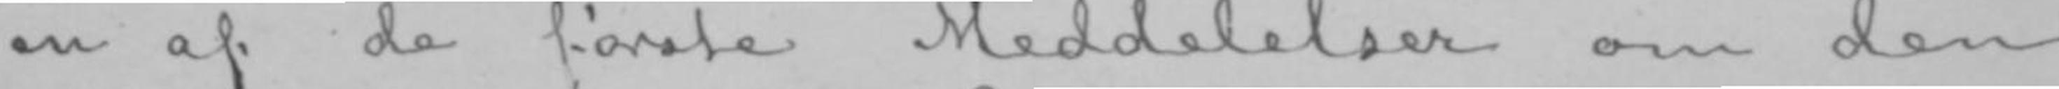en af de første Meddelelser om den
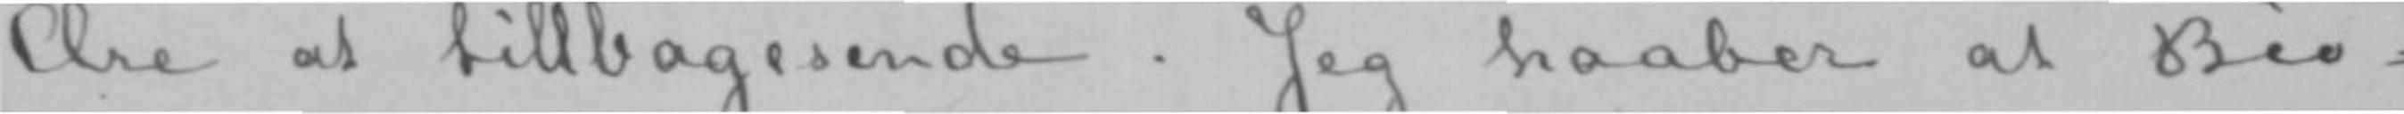Ære at tillbagesende. Jeg haaber at Bio-
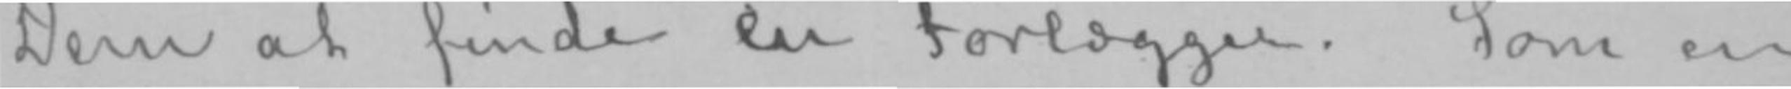Dem at finde en Forlægger. Som en
